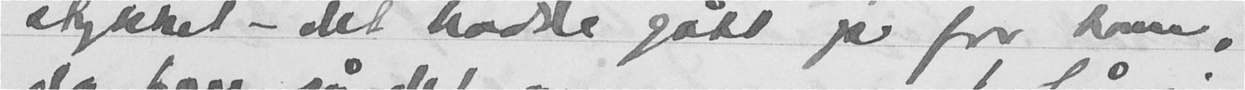stykket - det hadde gått op for ham,
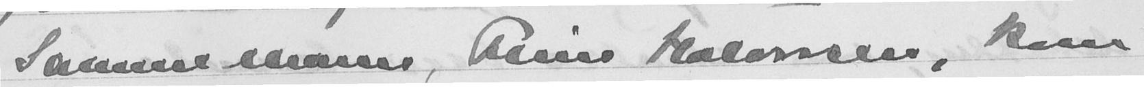Samme mann, Finn Halvorsen, kom
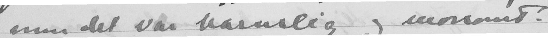men det var barnslig og morsomt.
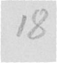18
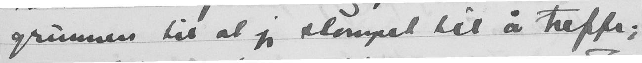grunnen til at jeg slompet til å treffe;
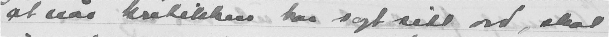at når kritikken har sagt sitt ord, skal
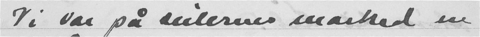Vi var på seilerens marked en
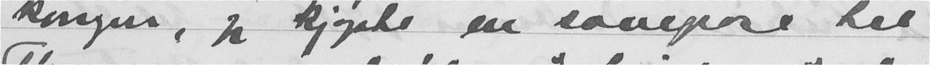Kongen, jeg kjøpte en sovepose til
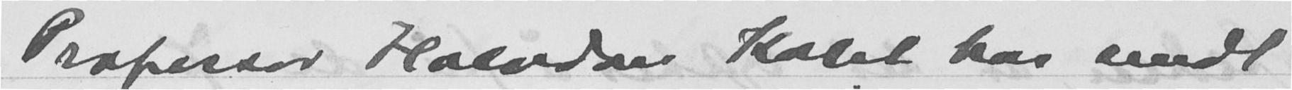Professor Halvdan Kolht har sendt
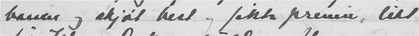banen og skjøt best og fikk premie, litt
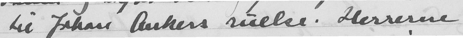til Johan Ankers ruelse. Herrerne
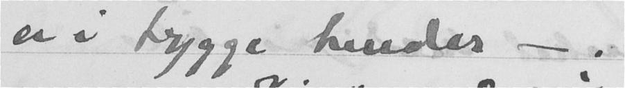er i trygge hender - .
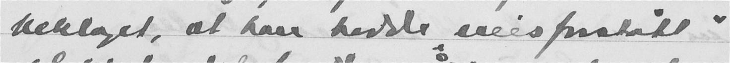beklaget, at han hadde "misforstått"
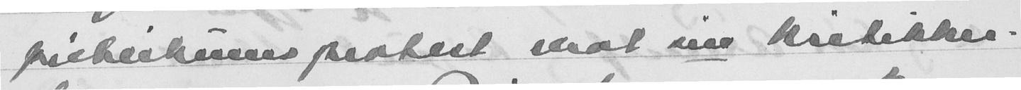publikumsprotest mot sine kritikker.
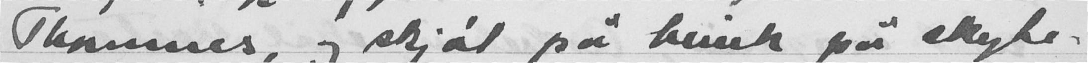Thommes, og skjøt på blink på skyte-
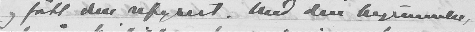og fått den refusert. Med den begrunnelse,
